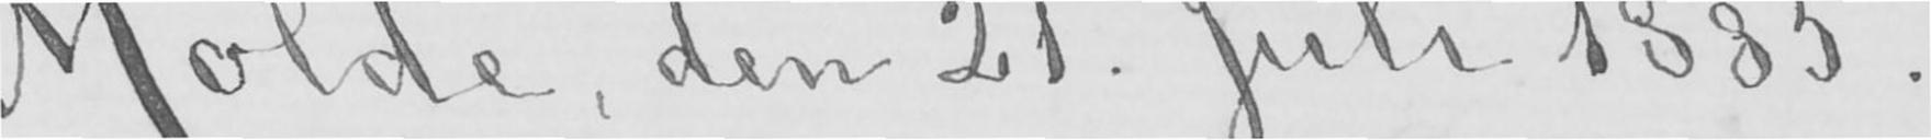Molde, den 21. Juli 1885.
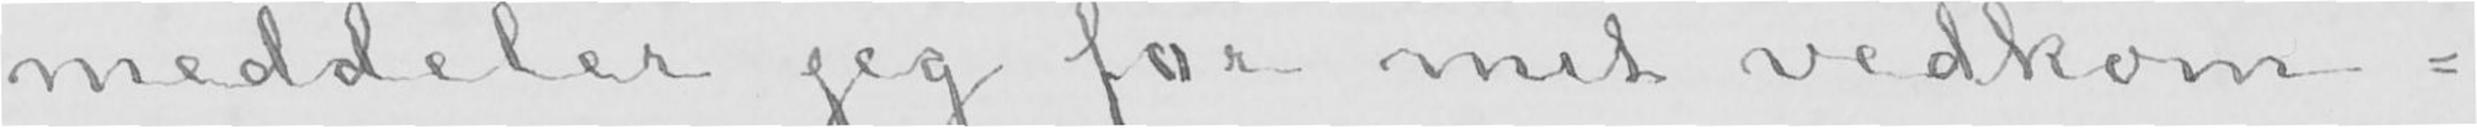meddeler jeg for mit vedkom-
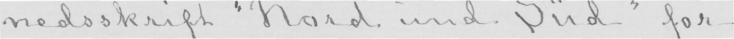nedsskrift «Nord und Süd» for
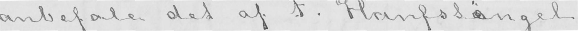anbefale det af F. Hanfstæängel
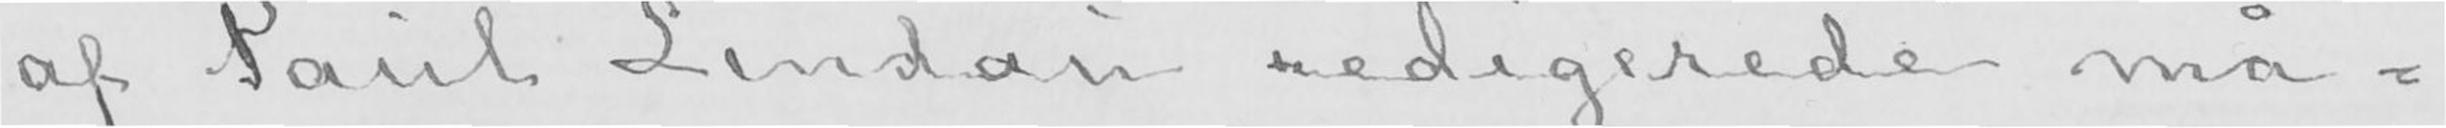af Paul Lindau redigerede må-
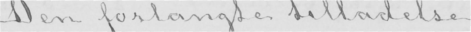Den forlangte tilladelse
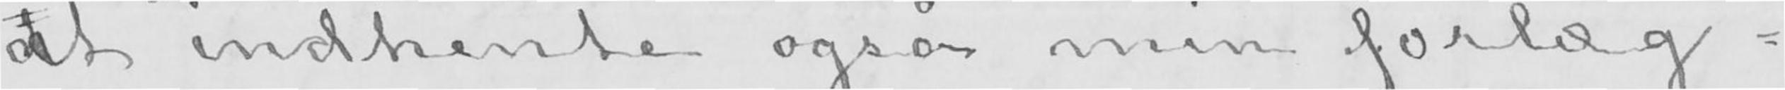at indhente også min forlæg-
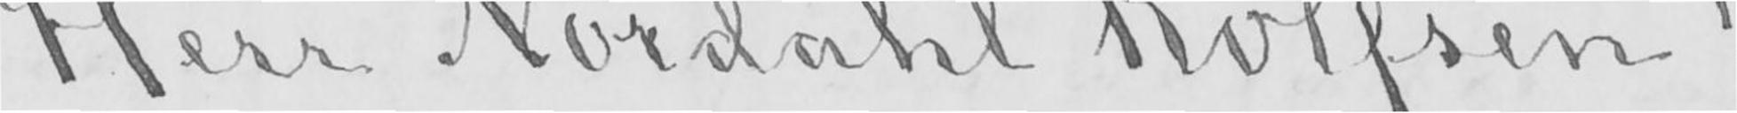Herr Nordahl Rolfsen!
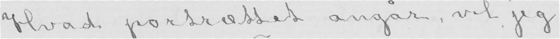Hvad portrættet angår, vil jeg
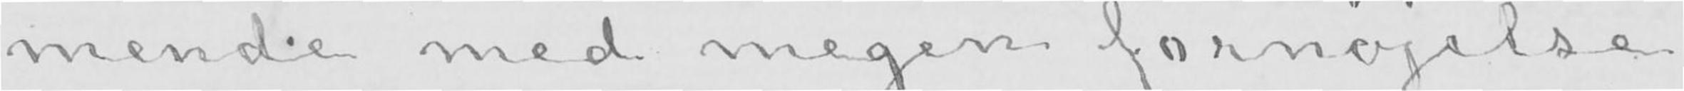mende med megen fornøjelse.
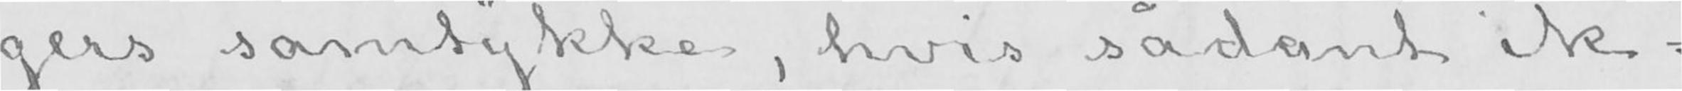gers samtykke, hvis sådant ik-
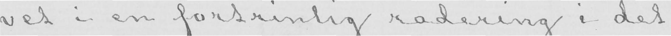vet i en fortrinlig radering i det
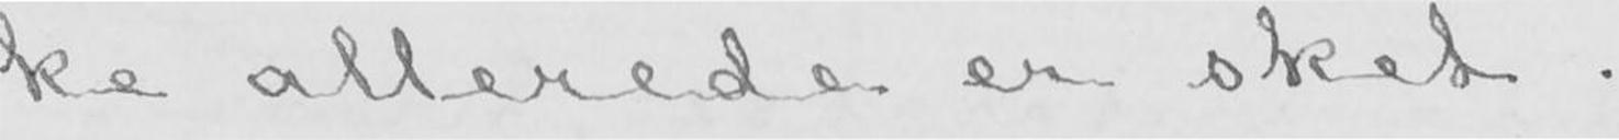ke allerede er sket.
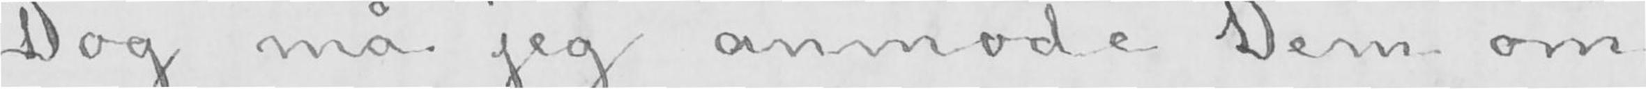Dog må jeg anmode Dem om
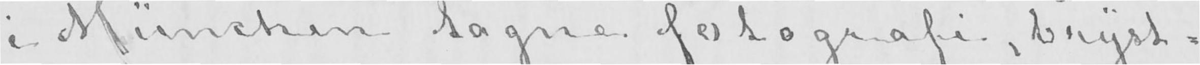i München tagne fotografi, bryst-
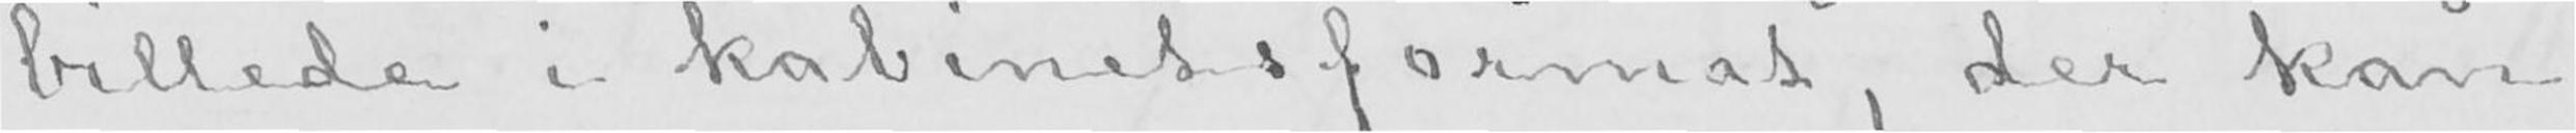billede i kabinetsformat, der kan
